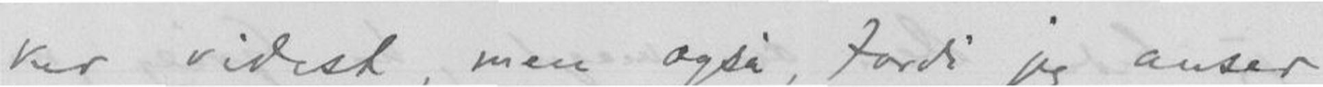ker videst, men også, fodi jeg anser
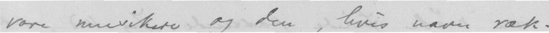vore musikere og den, hvis navn ræk-
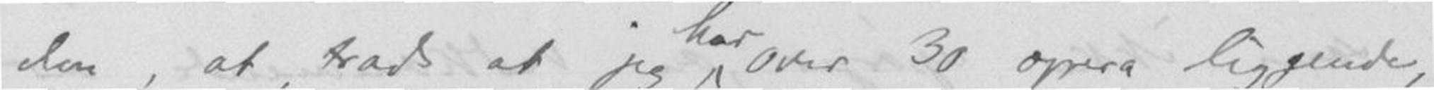den, at, trods at jeg har over 30 opera liggende,
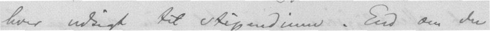hver udsigt til stipendium. End om du
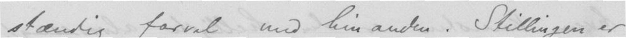stændig farvel med hinandem. Stillingen er
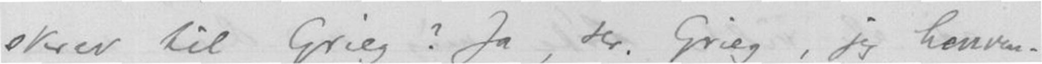skrev til Grieg? Ja, Hr. Grieg, jeg hænven-
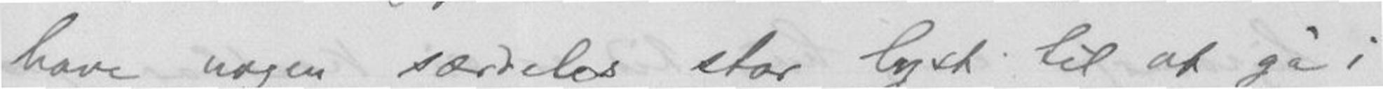have nogen særdeles stor lyst til at gå i
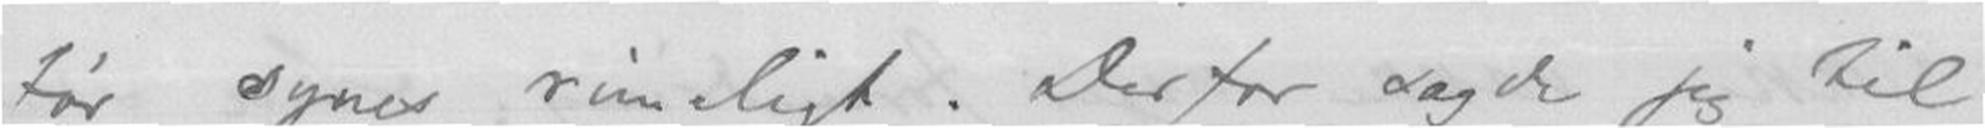tør synes rimeligt. Derfor sagde jeg til
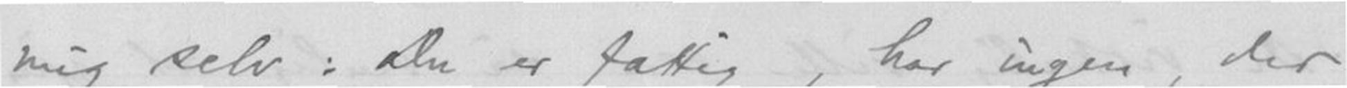mig selv: Du er fattig, har ingen, der
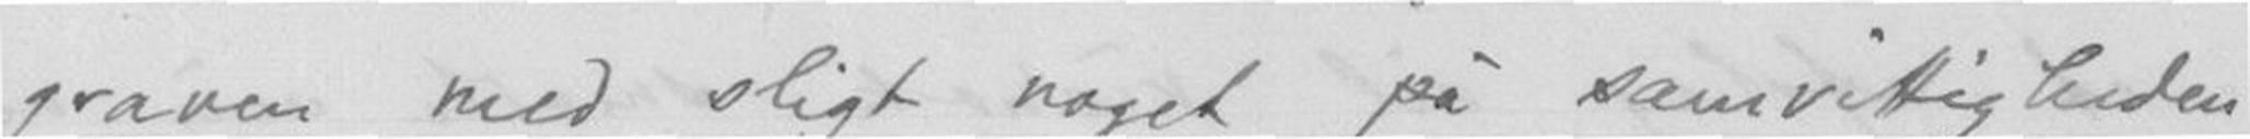graven med sligt noget på samvitigheden
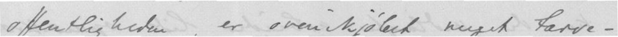offentligheden, er ovenikjøber meget tarve-
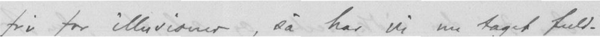fri for illusioner, så har vi nu taget fuld-
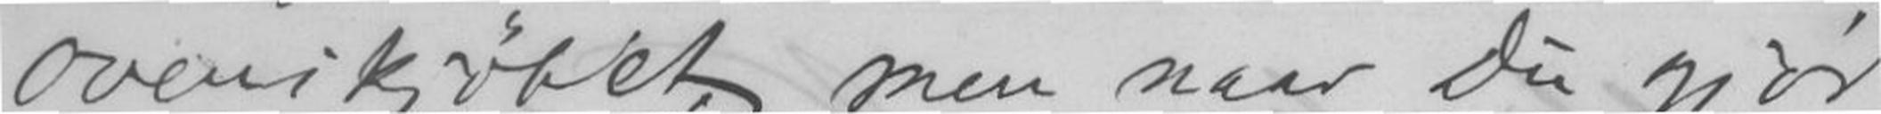ovenikjøbet, men naar Du gjør
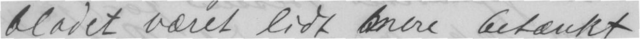blodet været lidt mere betenkt,
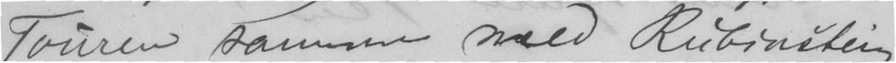Touren sammen med Rubinstein
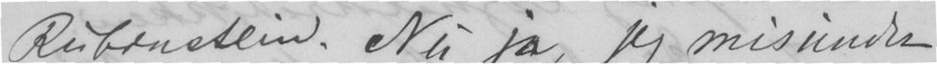Rubenstein. Nu ja, jeg misunder
